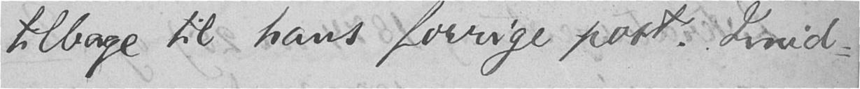tilbage til hans forrige post. Imid-
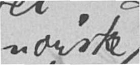norske
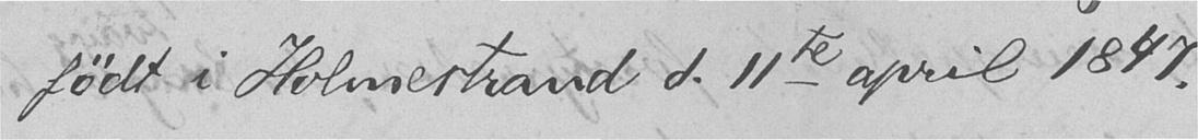født i Holmestrand d. 11te april 1847.
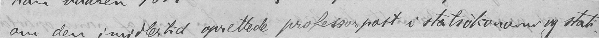om den imidlertid oprettede professorpost i statsøkonomi og stati-
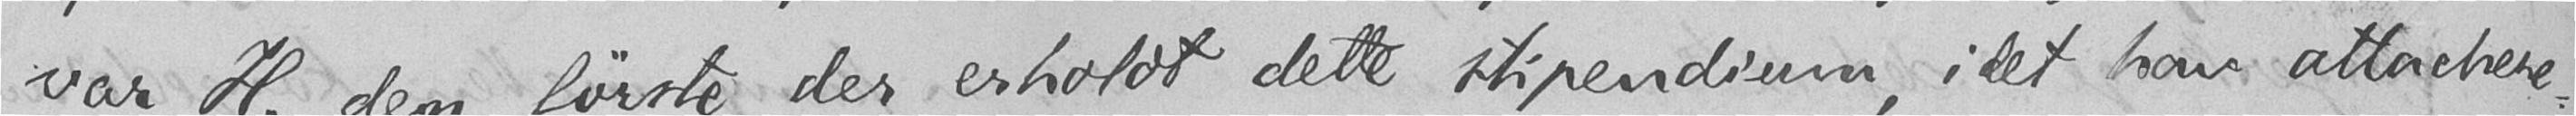var H. den første, der erholdt dette stipendium, idet han attachere-
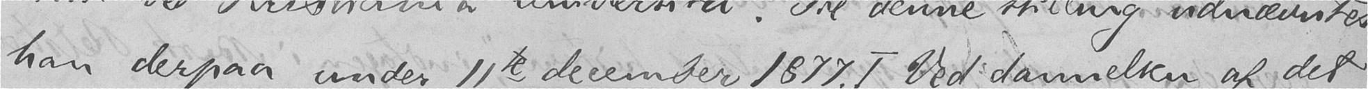han derpaa under 11te december 1877. I Ved dannelsen af det
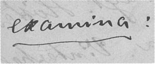examina:
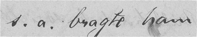s. a. bragte ham
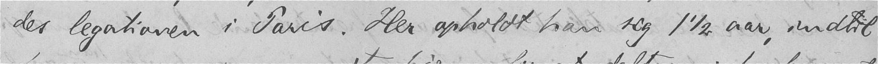des legationen i Paris. Her opholdt han sig 1 1/2 aar, indtil
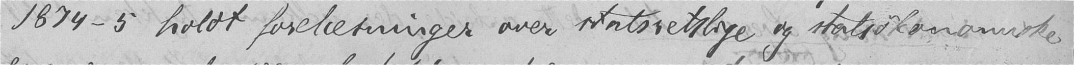1874-5 holdt forelæsninger over statsretslige og statsøkonomiske
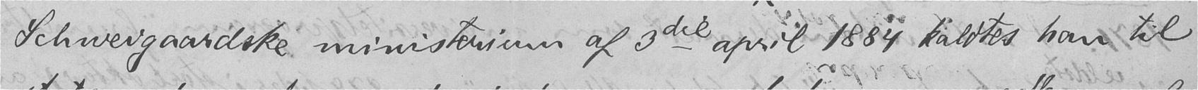Schweigaardske ministerium af 3die april 1884 kaldtes han til
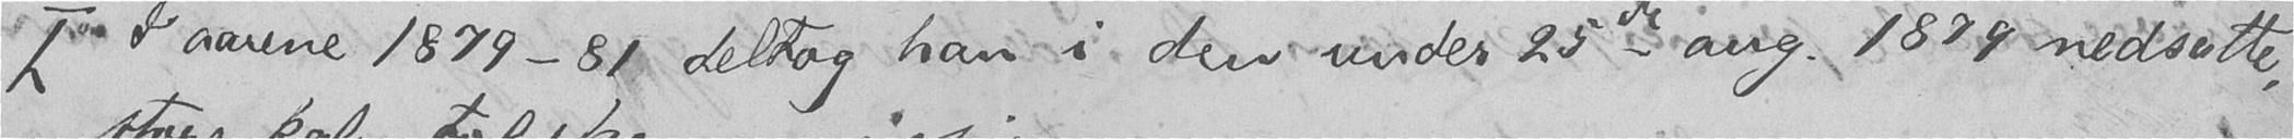I I aarene 1879-81 deltog han i den under 25de aug. 1879 nedsatte,
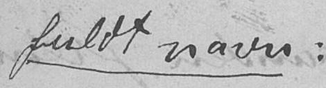fuldt navn:
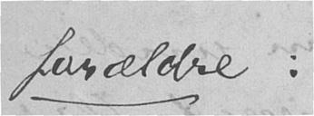forældre:
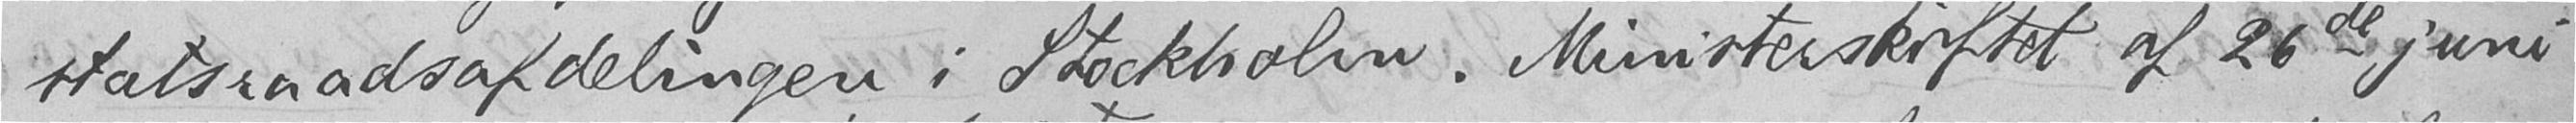statsraadsafdelingen i Stockholm. Ministerskiftet af 26de juni
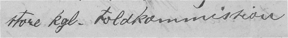store kgl. toldkommission.
